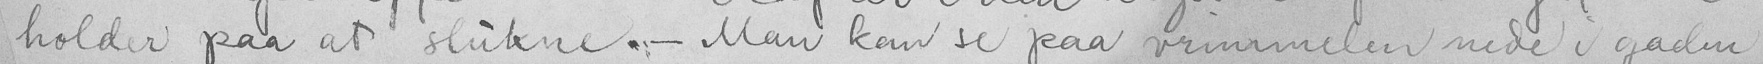holder paa at slukne. - Man kan se paa vrimmelen nede i gaden
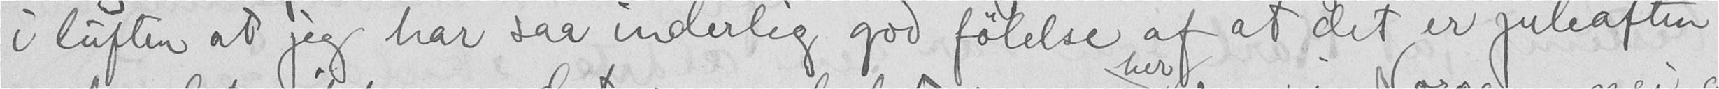i luften at jeg har saa inderlig god følelse af at det er juleaften
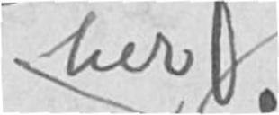her
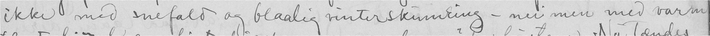ikke med snefald og blaalig vinterskumring, - nei men med varm
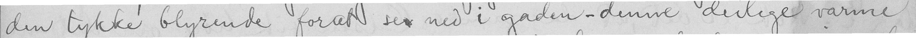den tykke blyrende forat se ned i gaden - denne deilige varme
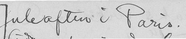Juleaften i Paris.
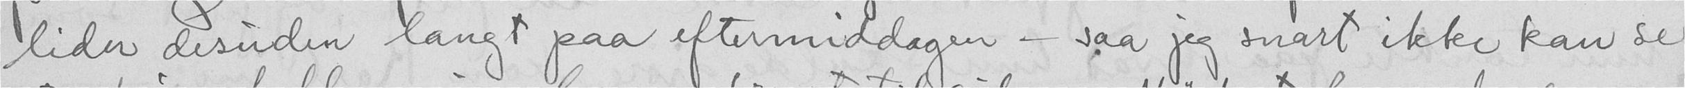lider disuden langt paa eftermiddagen - saa jeg snart ikke kan se
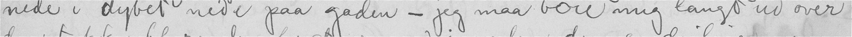nede i dybet nede paa gaden - jeg maa bøie mig langt ud over
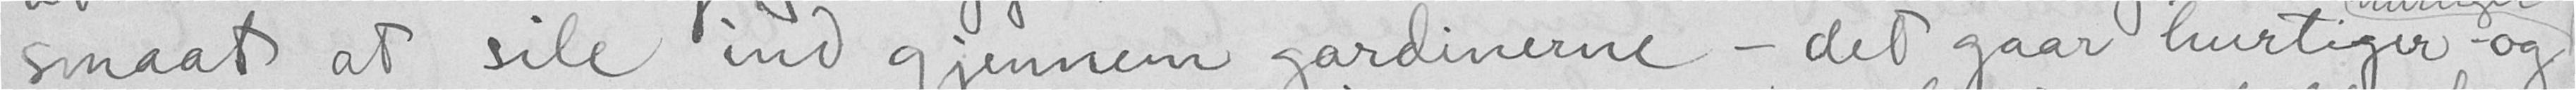smaat at sile ind gjennem gardinerne - det gaar hurtiger og
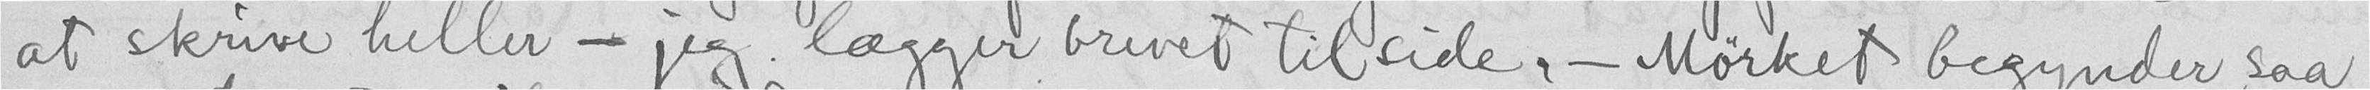at skrive heller - jeg lægger brevet tilside. - Mørket begynder saa
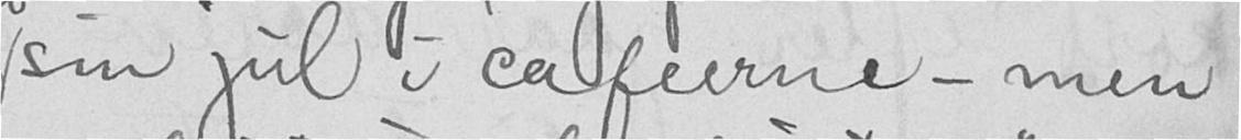sin jul i cafeerne - men
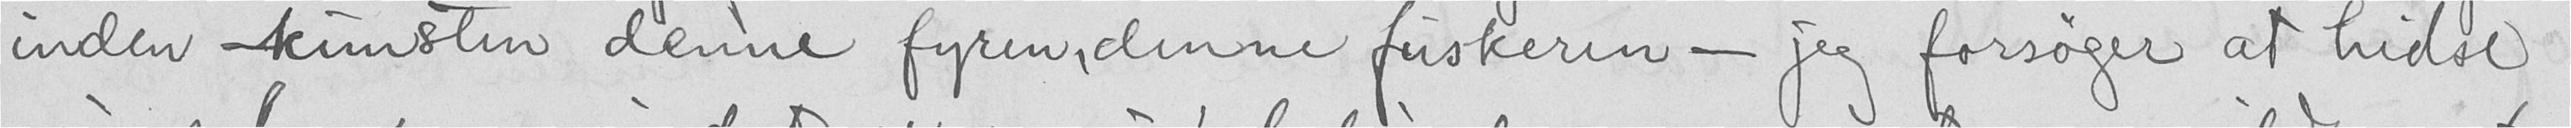inden kunsten denne fyren, denne fuskeren - jeg forsøger at hidse
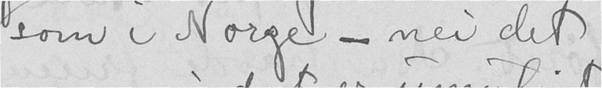som i Norge, - nei det
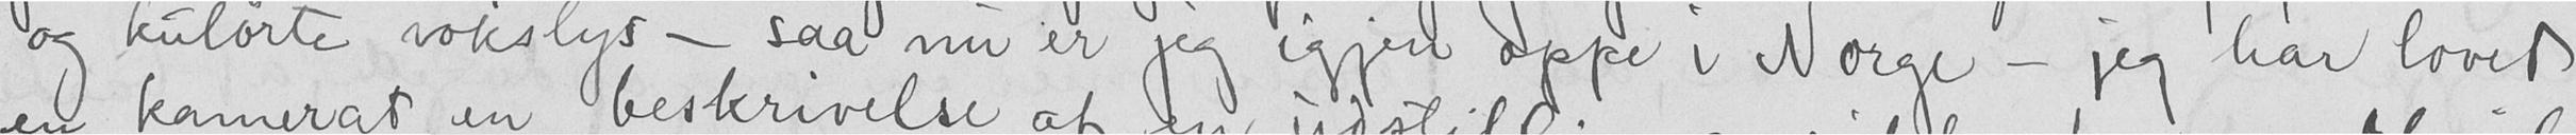og kulørte vokslys - saa nu er jeg igjen oppe i Norge - jeg har lovet
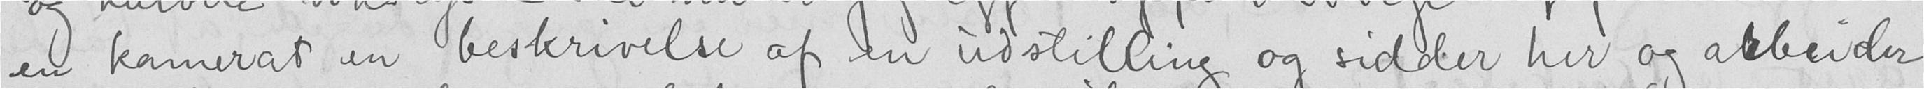en kamerat en beskrivelse af en udstilling og sidder her og arbeider
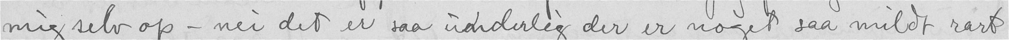mig selv op - nei det er saa underlig der er noget saa mildt rart
